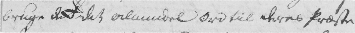bruge de det almindel Ord til deres Præste
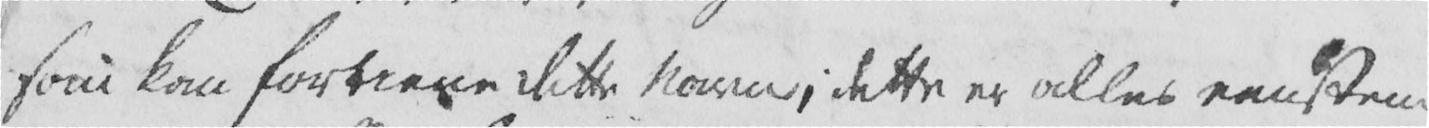som kan fortiene dette Navn; dette er alles eenstem-
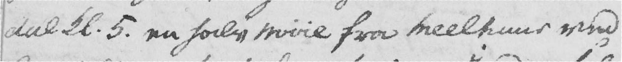dal kl. 5. en halv Miil fra Melhuus ved
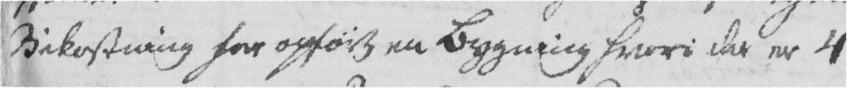Bekostning har opført en Bygning hvori der er 4
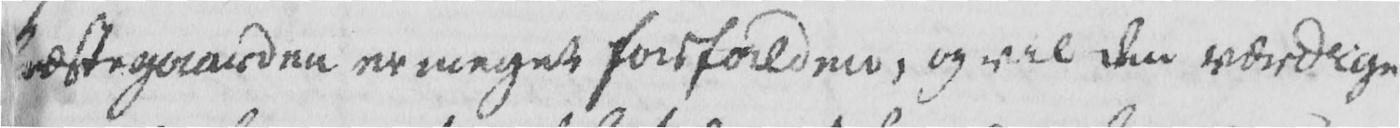Præstegaaarden er meget forfalden, og vil den værdige
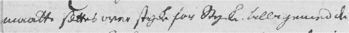maatte sættes over stycke for Stycke tilligemed de
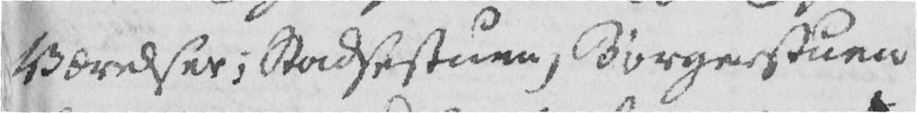værelser; Stadsestuen, Borgerstuen
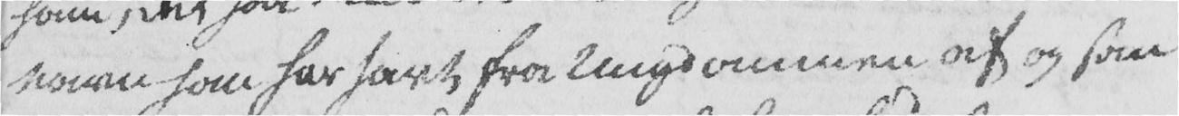Navn han har havt fra Ungdommen af og som
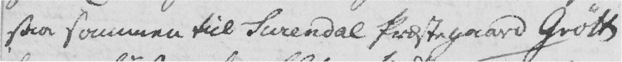saa sammen til Surendal Præstegaard Grøtte
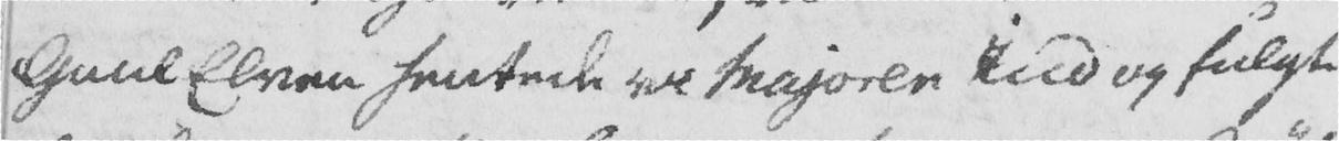Guul Elven hentede vi Majoren ind og fulgte
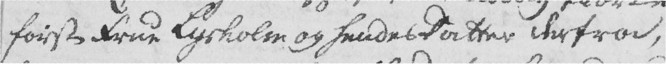først Frue Lysholm og hendes Datter derfra,
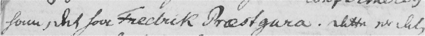ham, det har Fredrik Præstgara. Dette er det
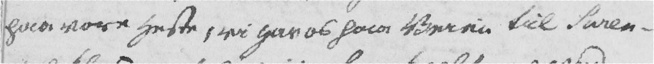paa vore Heste, vi gav os paa Veien til Suren-
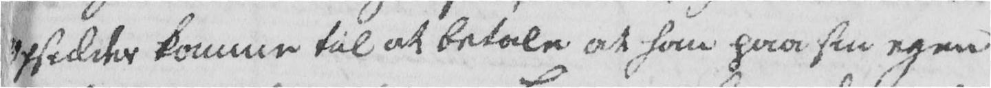Opsidder komme til at betale at han paa sin egen
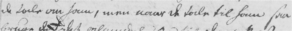de tale om ham, men naar de tale til ham saa
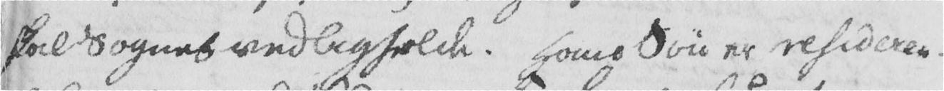skal Sognet vedligeholde. Hans Søn er resideren-
